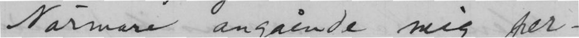Närmare angående mig per-
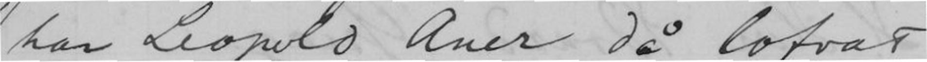har Leopold Auer då lofvat
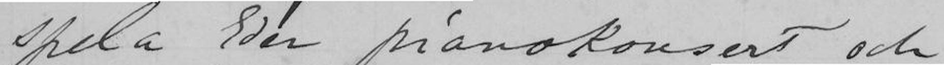spela Eder pianokonsert och
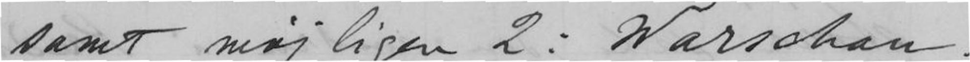samt möjligen 2: Warschau.
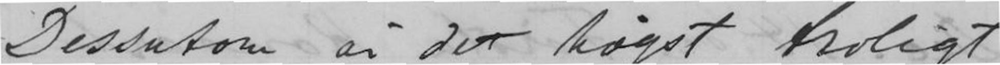Dessutom är det högst troligt
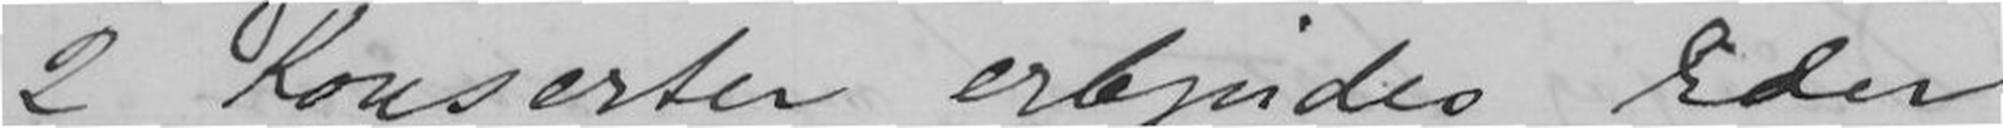2 Konserter erbjudes Eder
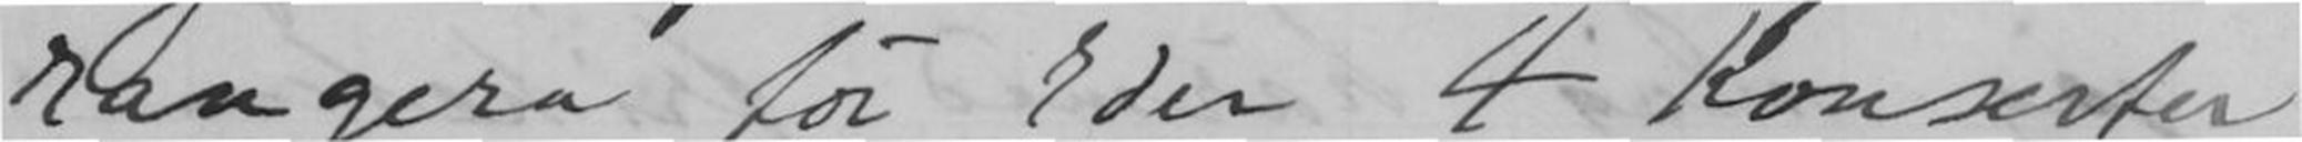rangera för Eder 4 Konserter
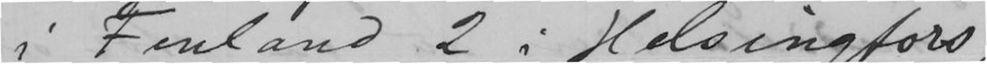i Finland 2 i Helsingfors,
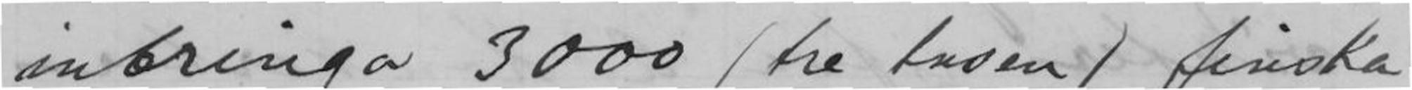inbringa 3000 (tre tusen) finska
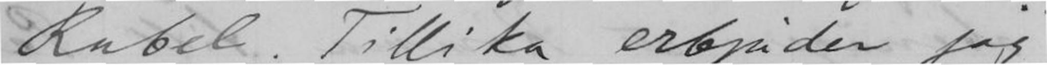Rubel. Tillika erbjuder jag
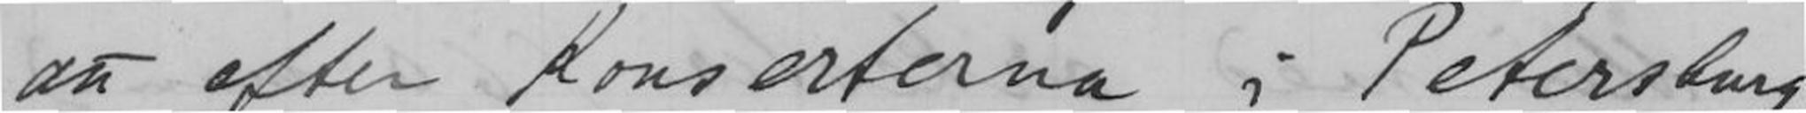att efter konserterna i Petersburg
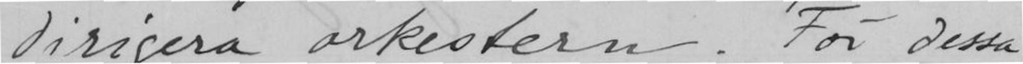dirigera orkestern. För dessa
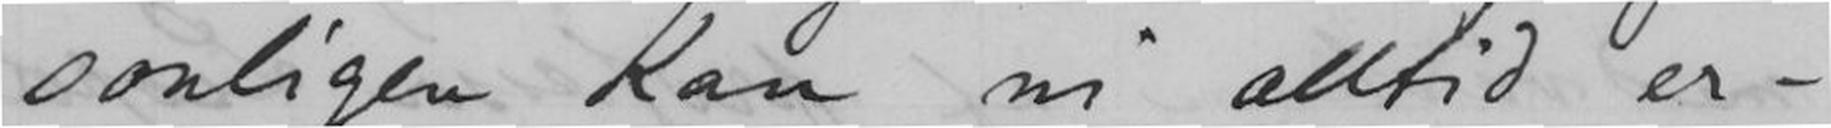sonligen kan ni alltid er-
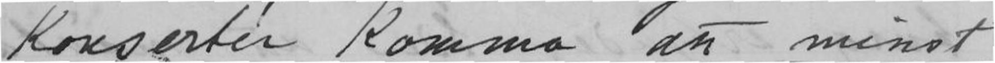konserter komma att minst
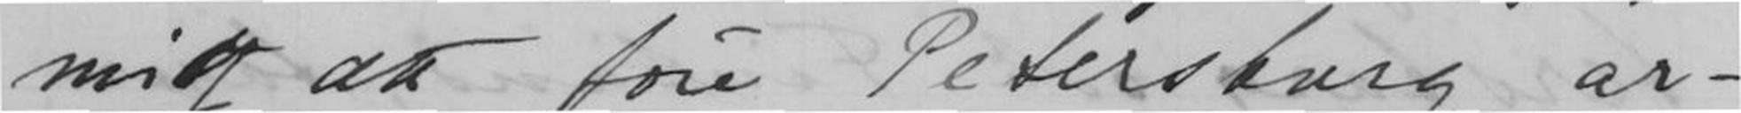mig att före Petersburg ar-
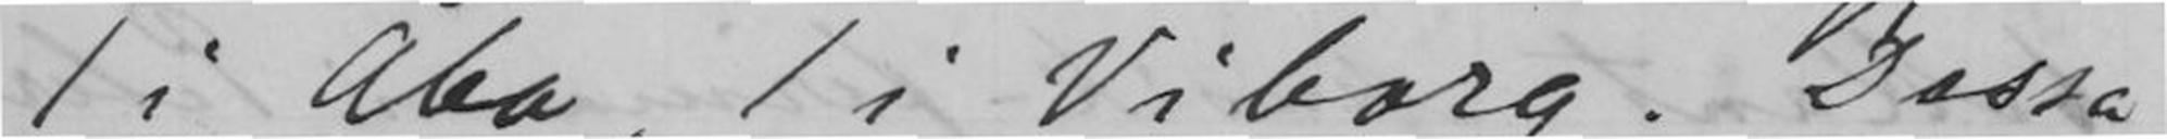1 i Åbo, 1 i Viborg. Dessa
